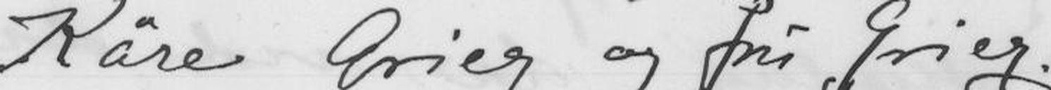Käre Grieg og fru Grieg.
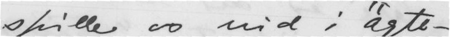spille os ind i ägtes-
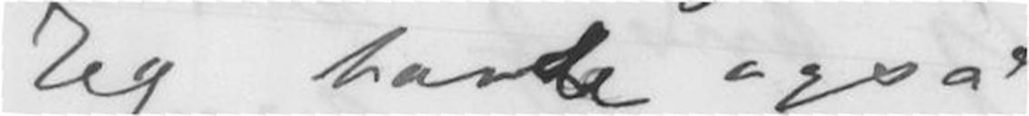ord! Jeg havde også
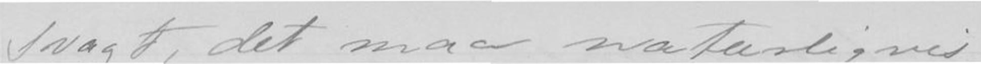svagt, det maa naturligvis
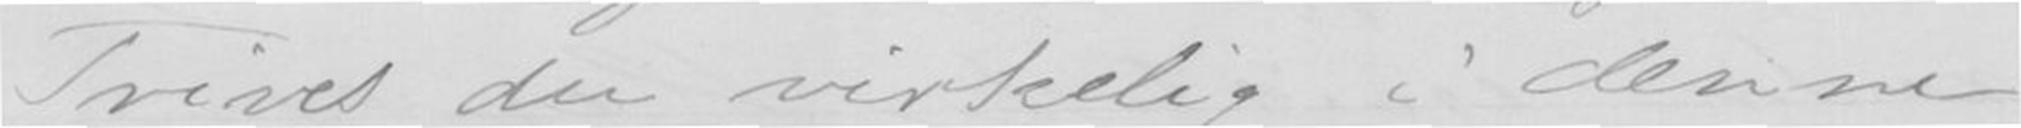Trives du virkelig i denne
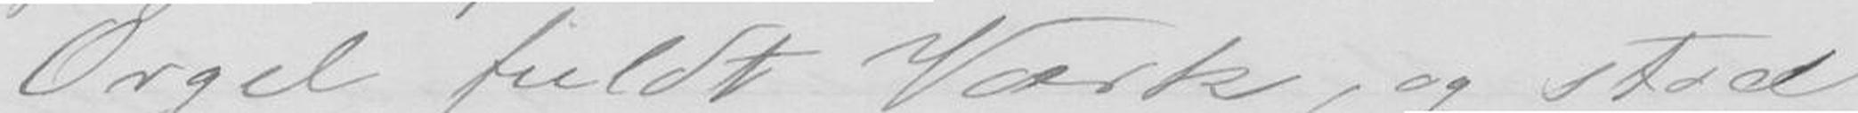Orgel fuldt Værk, og stod
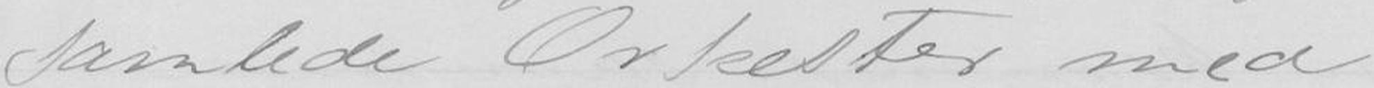samlede Orkester med
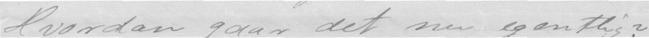Hvordan gaar det nu egentlig?
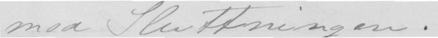mod Sluttningen. -
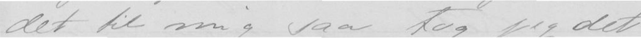det til mig saa tog jeg det
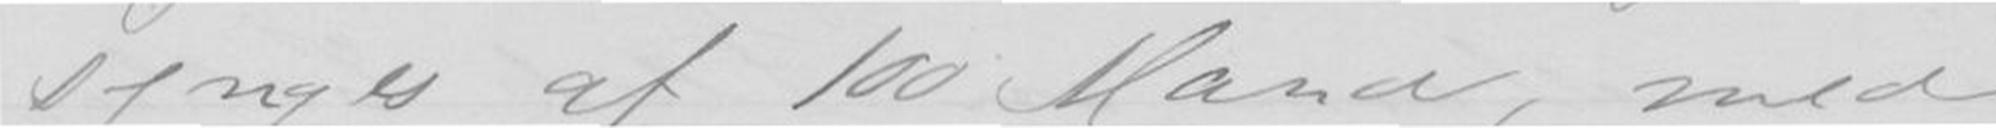synges af 100 Mand, med
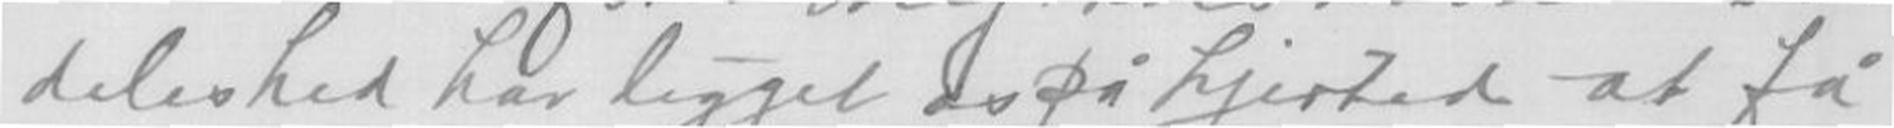deleshed har ligget os på hjerted at få
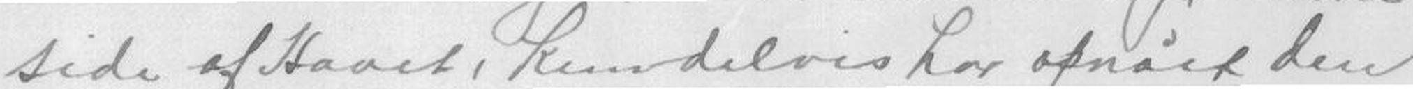Side af Havet, kun delvis har opnået den
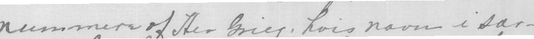nummere af Her Grieg, hvis navn i sær-
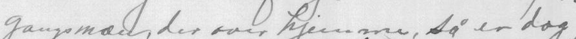gangsmæn, der over hjemme, så er dog
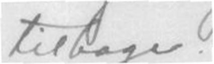tilbage.
